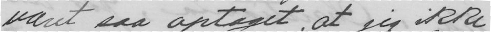været saa optaget, at jeg ikke
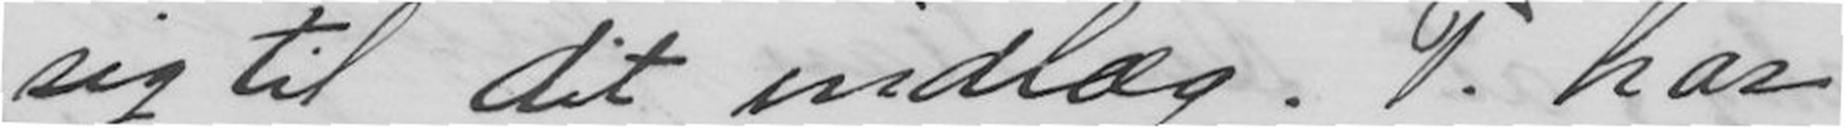sig til dit indlæg. T. har
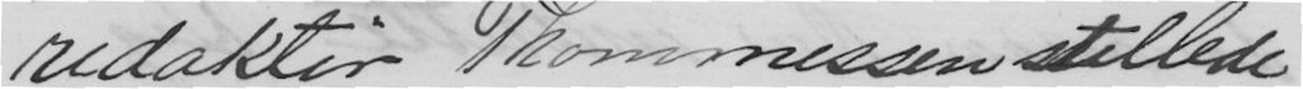redaktør Thommessen stillede
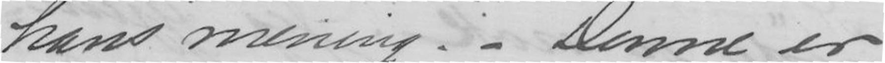hans mening. - Denne er
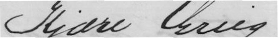Kjære Grieg
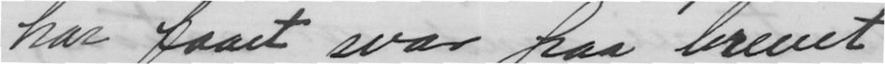har faaet svar paa brevet
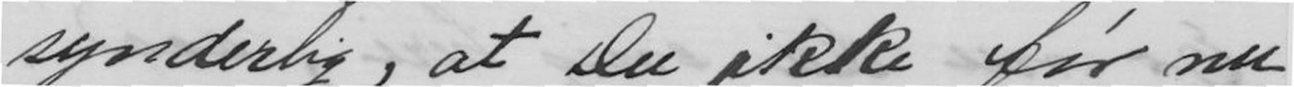synderlig, at Du ikke før nu
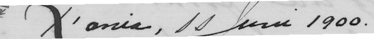X'ania, 11 Juni 1900.
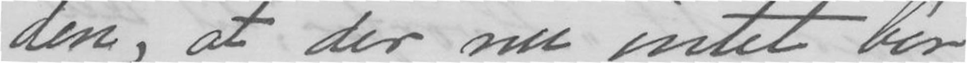den, at der nu intet bør
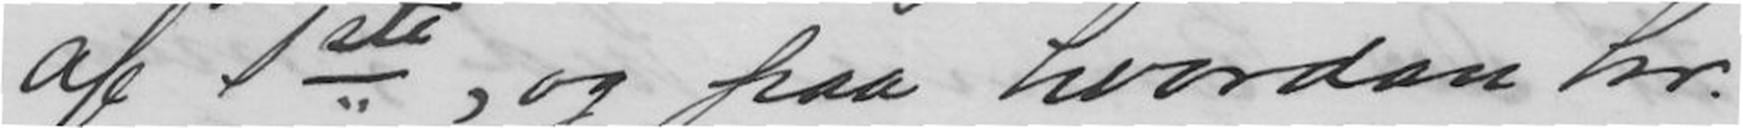af 1ste, og paa hvordan hr.
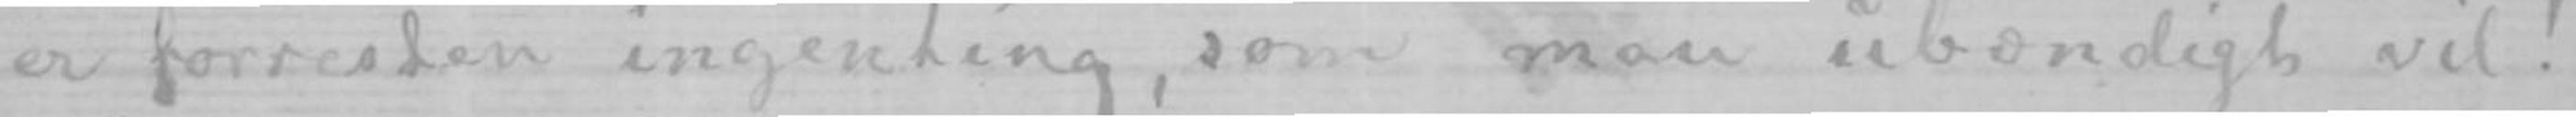er forresten ingenting, som man ubændigt vil!
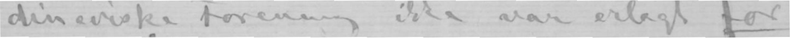dinaviske Forening ikke var erlagt for-
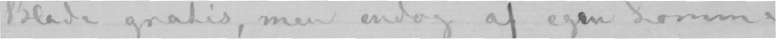Blade gratis, men endog af egen Lomme
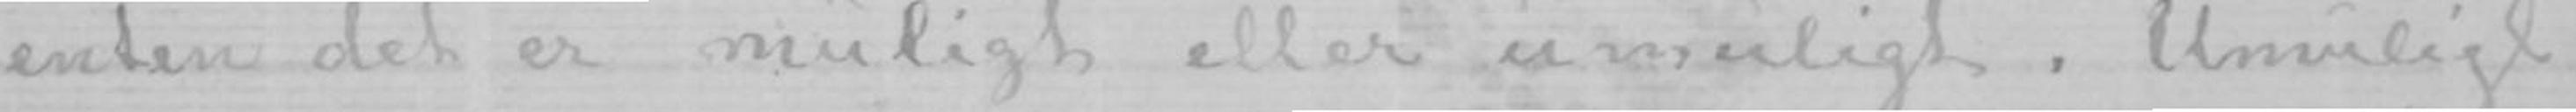enten det er muligt eller umuligt. Umuligt
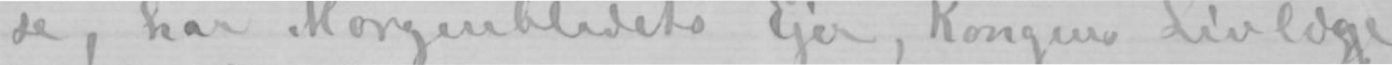se, har Morgenbladets Ejer, Kongens Livlæge
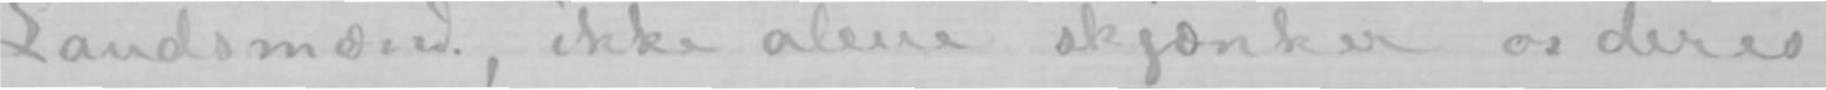Landsmænd, ikke alene skjænker os deres
